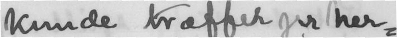jeg kunde træffe jer her-
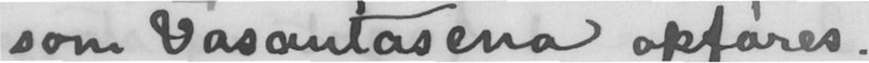som Vasantasena opføres.
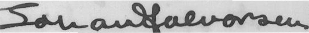Johan Halvorsen
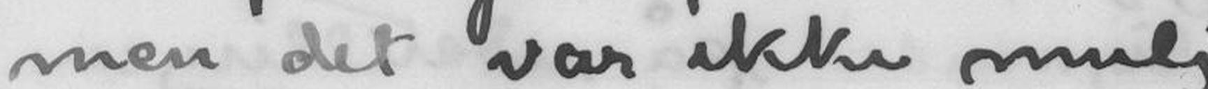men det var ikke mulig
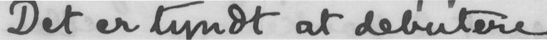Det er tyndt at debutere
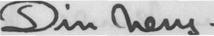Din heng.
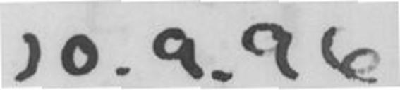10.9.96
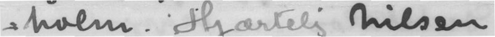holm. Hjærtelig hilsen
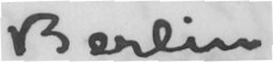Berlin
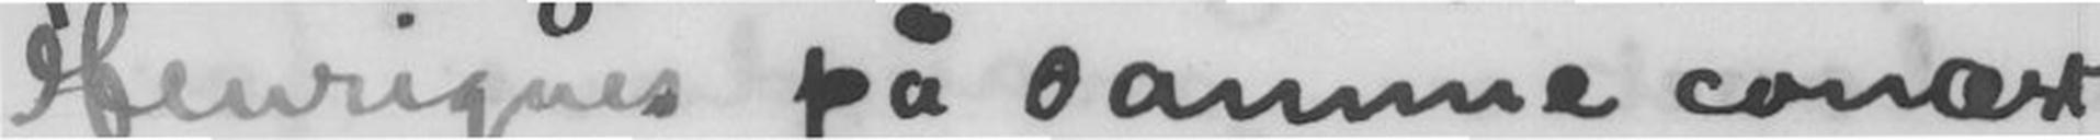Henriques på samme concert
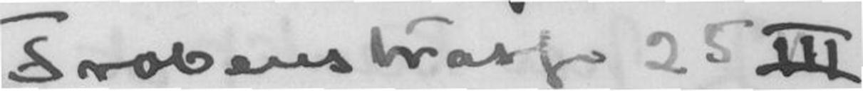Frobaustrasse 25 III
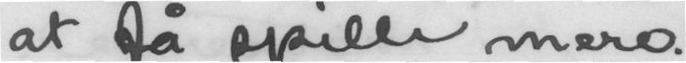at få spille mere.
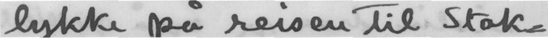lykke på reisen til Stok-
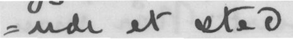ude et sted.
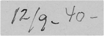12/9. 40.
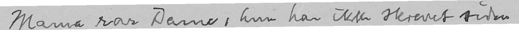Mama rar Dame, hun har ikke skrevet siden
